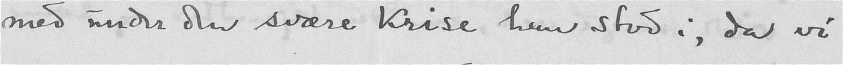med under den svære krise han stod i, da vi
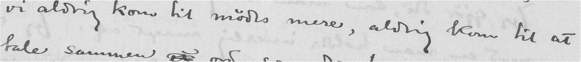vi aldrig kom til mødes mere, aldrig kom til at
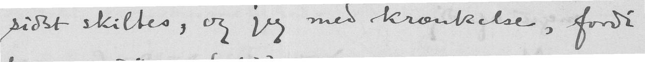sidst skiltes, og jeg med krænkelse, fordi
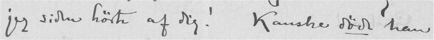jeg siden hørte af dig! Kanske døde han
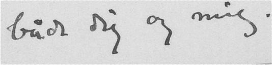både dig og mig.
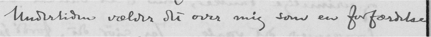Undertiden vælder det over mig som en forfærdelse
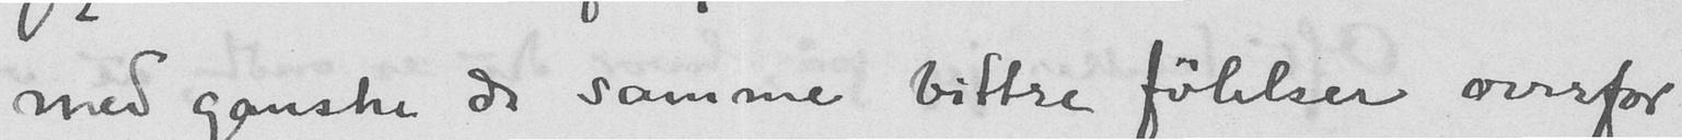med ganske de samme bittre følelser overfor
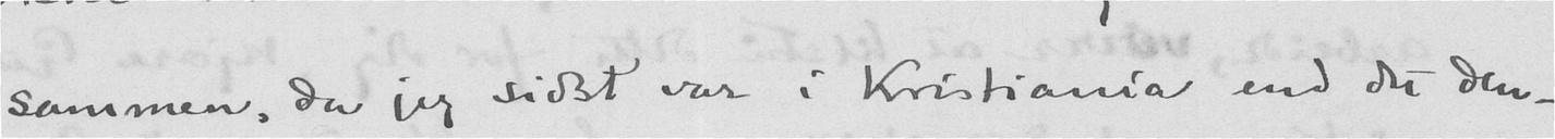sammen, da jeg sidst var i Kristiania end det den-
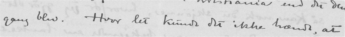gang blev. Hvor let kunde det ikke hændt, at
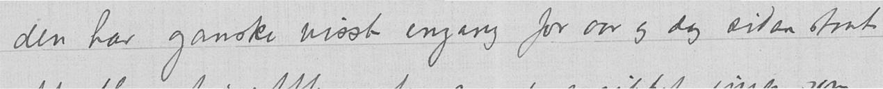den har ganske visst engang for aar og dag siden staat
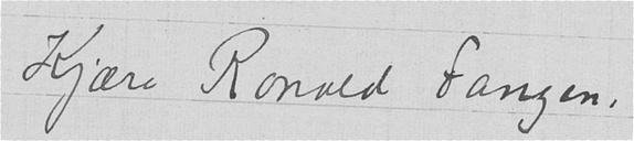Kjære Rohald Fangen,
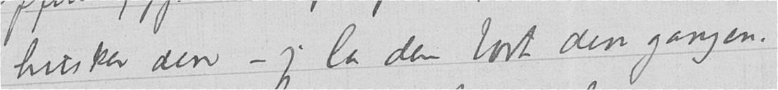husker den – jeg la den bort den gangen.
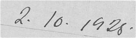2.10.1928.
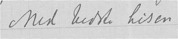Med bedste hilsen
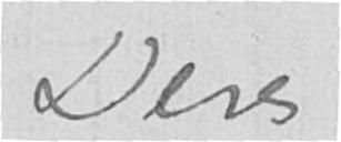Deres
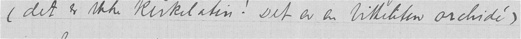(det er ikke kirkelatin! Det er en bitteliten orchide)
